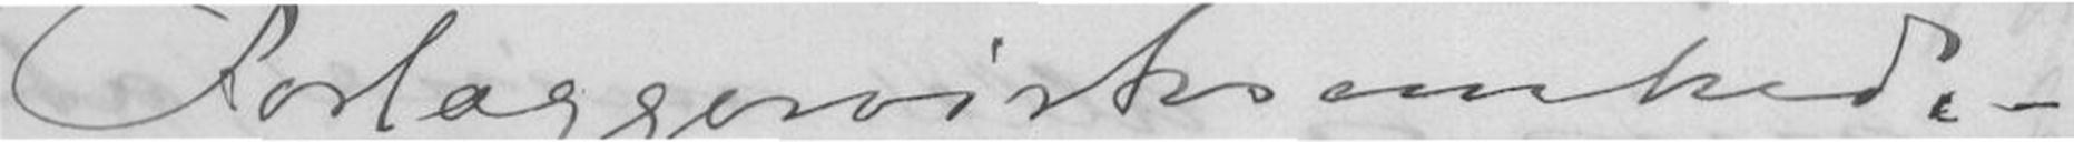Forlæggervirksomhed. -
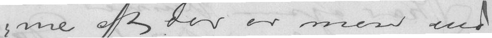me at der er mere end
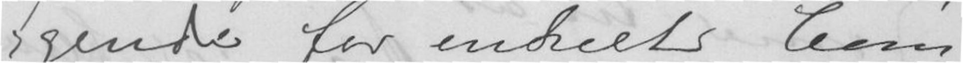gende for enkelte Com-
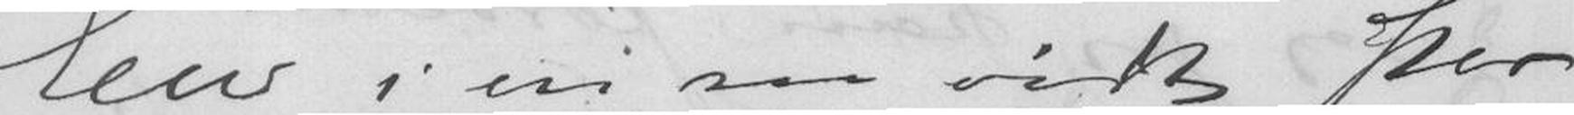Ene i en saa vidt stor
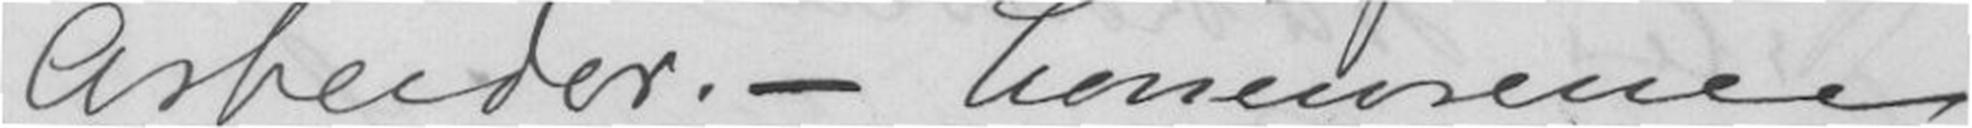Arbeider. - Cocurance
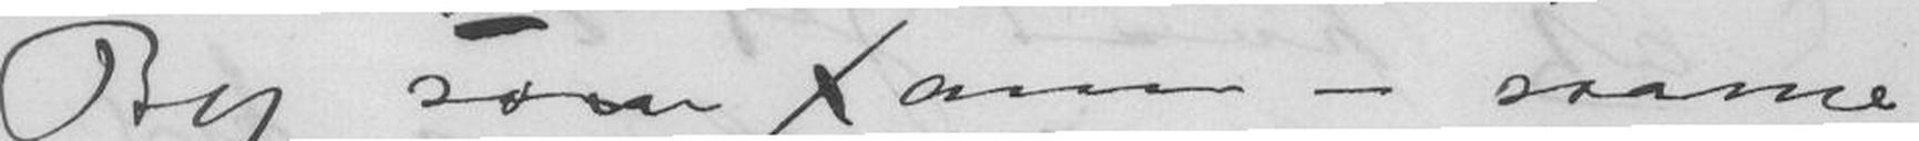By som Xania - saame-
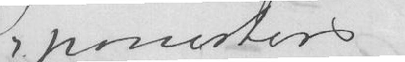ponister
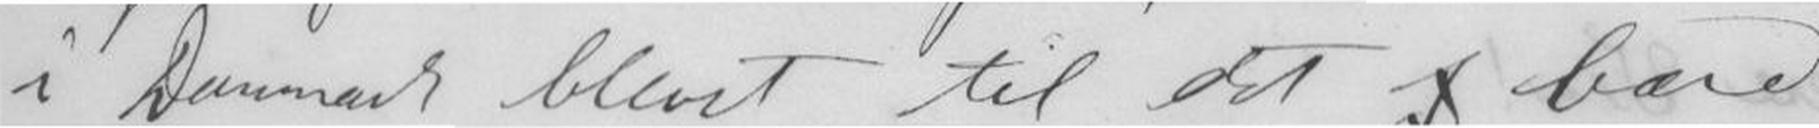i Danmark blevet til det bare
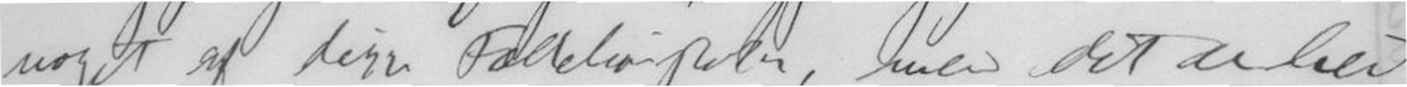noget af disse Folkehøiskoler, men det er her
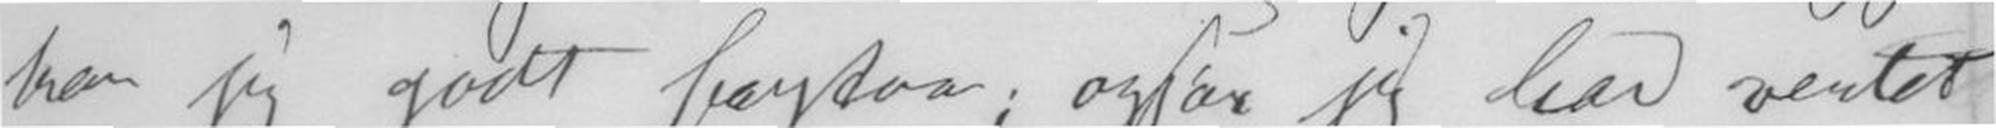kan jeg godt forstaa; ogsaa jeg har ventet
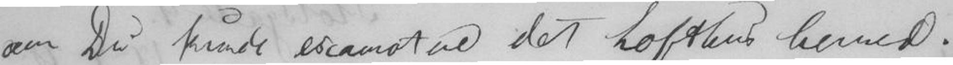om Du kunde escamotere det Lofthus herned.
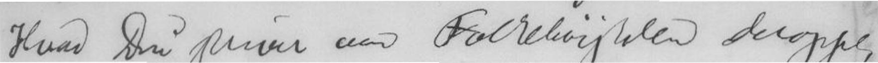Hvad Du skriver om Folkehøiskolen deroppe,
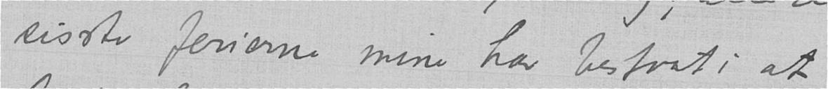sisste ferierne mine har bestaat i at
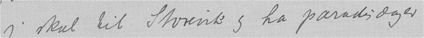jeg skal til Storevik og ha paradisdager
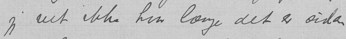- jeg vet ikke hvor længe det er siden
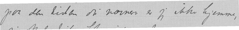paa den tiden du nævner er jeg ikke hjemme,
